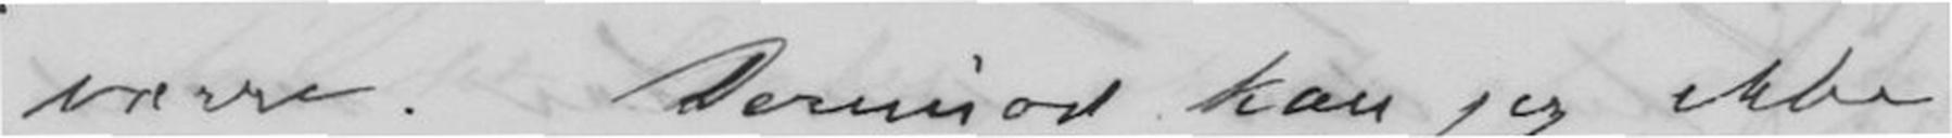værre. Derimod kan jeg ikke
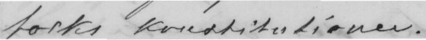folks konstitutioner.
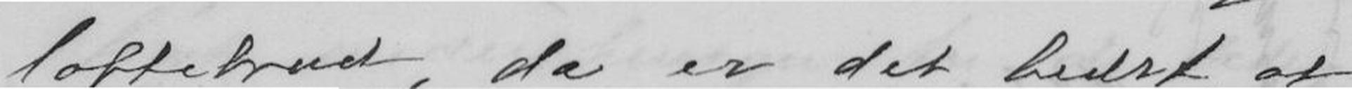loftebrud, da er det bedst, at
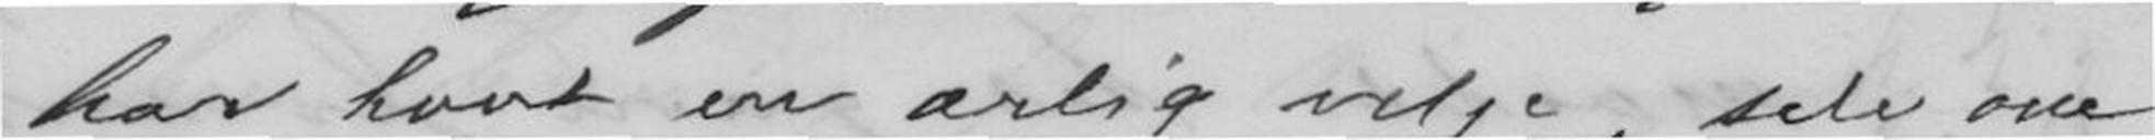har havt en ærlig vilje, selv om
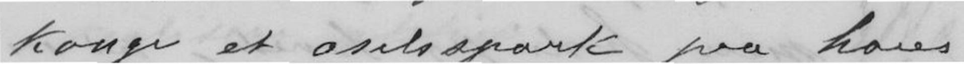konge et æselsspark paa hans
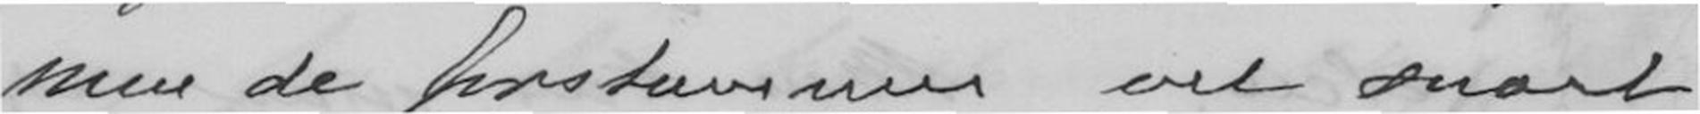men de forstummer vel snart
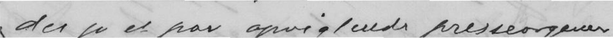der jo et par opviglende presseorganer,
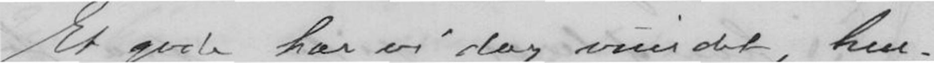Et gode har vi dog vundet, hen-
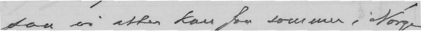saa vi atter kan faa sommer i Norge.
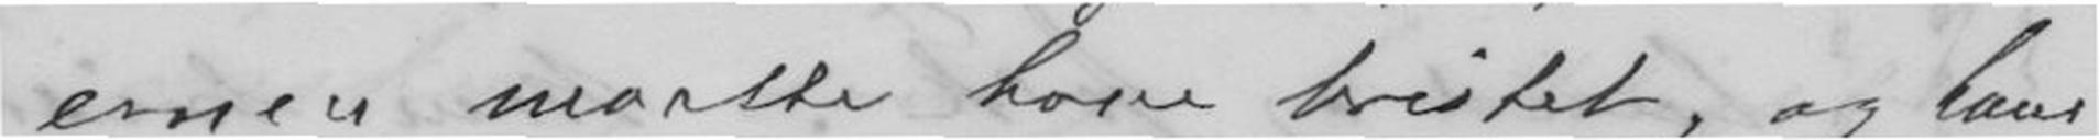evnen maatte have bristet, og hans
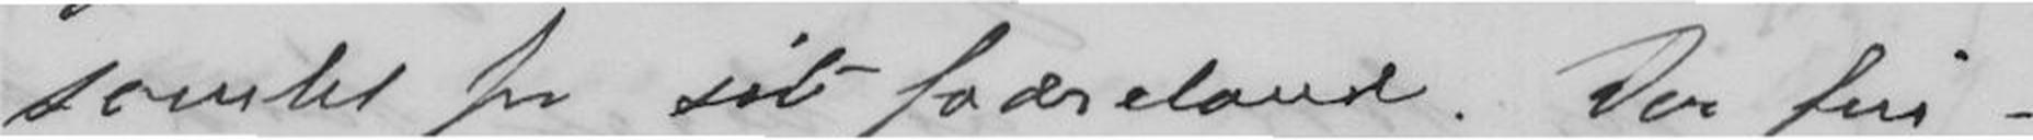samlet for sit fædreland. Der fin-
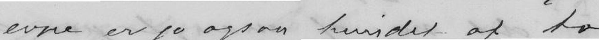evne er jo ogsaa bundet af to
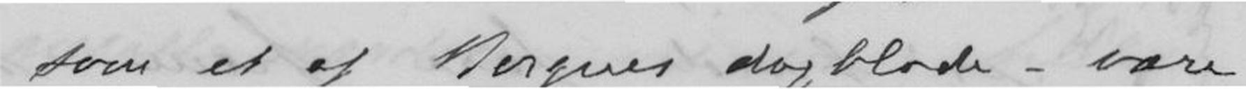- som et af Bergens dagblade - være
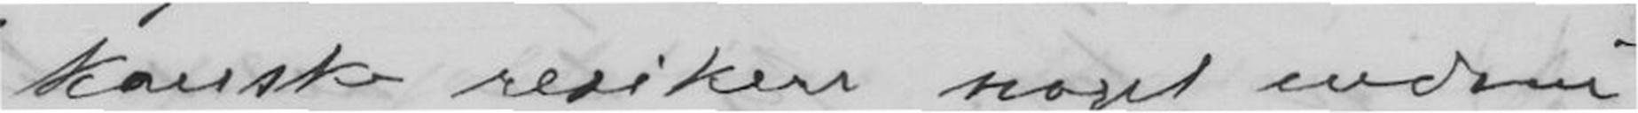kanske resikere noget endnu
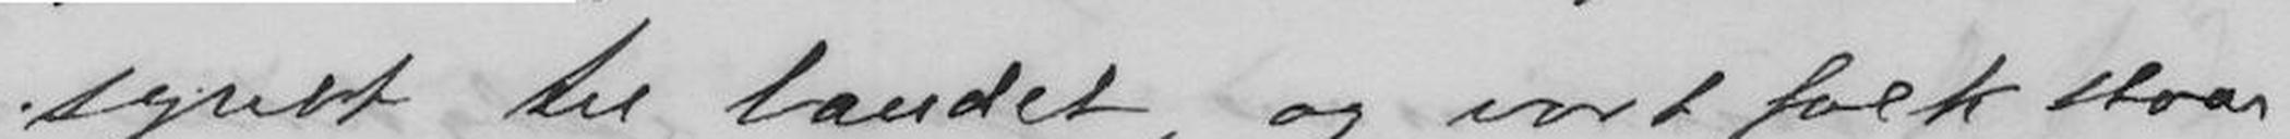synet til landet, og vort folk staar
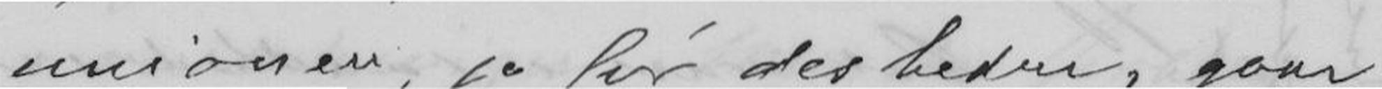unionen, jo før desbedre, gaar
